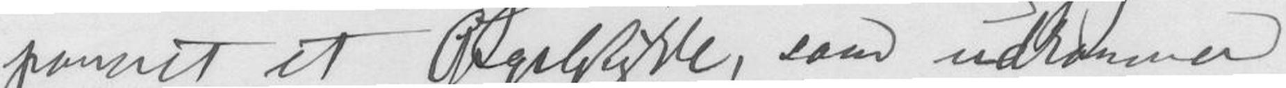poneret et Orgelstykke, som udkommer
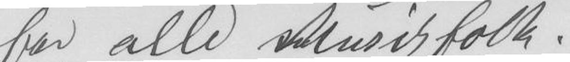for alle Musikfolk.
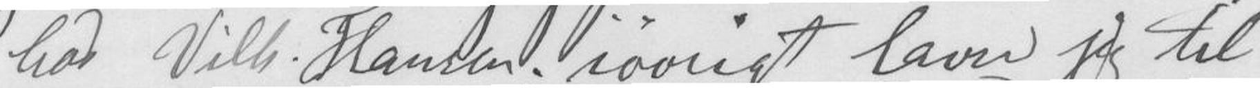hos Vilh. Hansen; iøvrigt laver jeg til
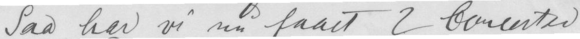Saa har vi nu faaet 2 Concerter
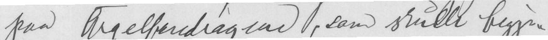paa Orgelforedragene, som skulle begyn-
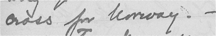cross for Norway. -
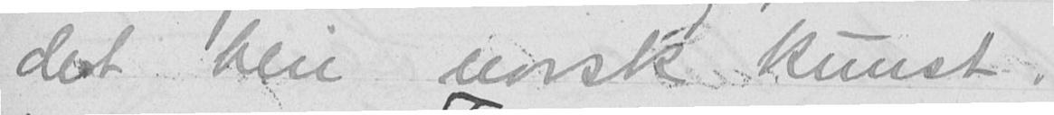det blir norsk kunst.
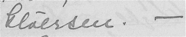Gløersen. -
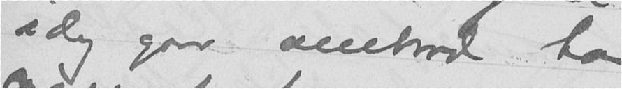idag gaar ombord to
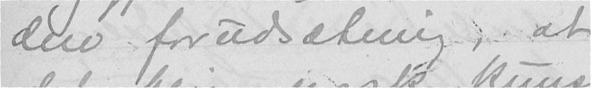den forudsætning, at
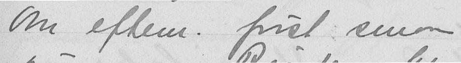Om efterm. først smaa
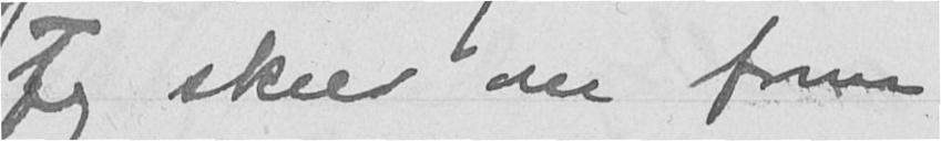Jeg skrev om form
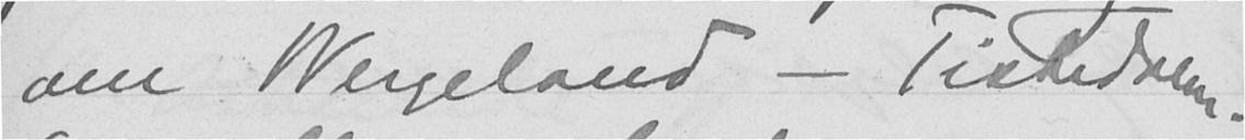om Wergeland - Tistedalen.
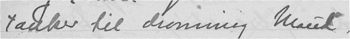tanker til dronning Maud,
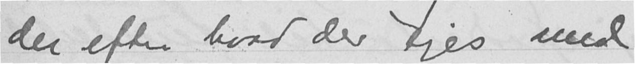der efter hvad der siges med
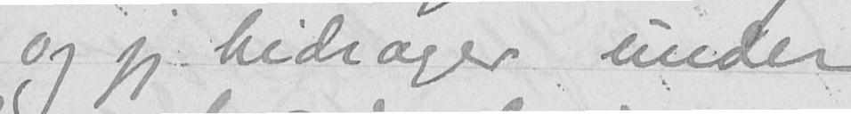og jeg bidrager under
